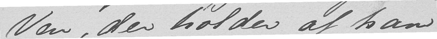Ven, der holder af ham
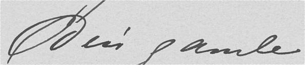Din gamle
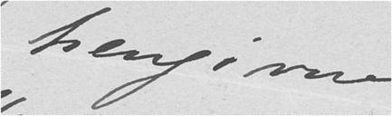hengivne
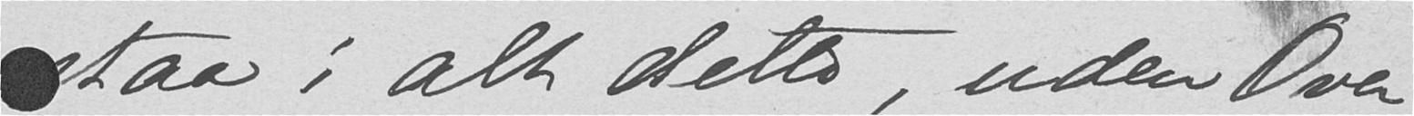staa i alt dette, uden Over-
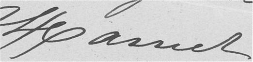Harriet
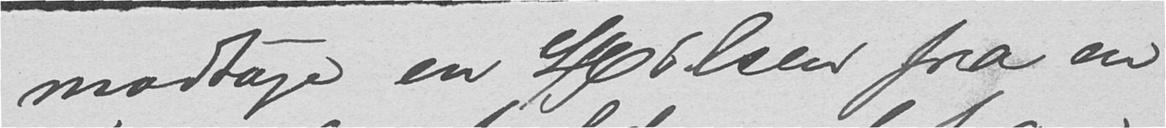modtage en Hilsen fra en
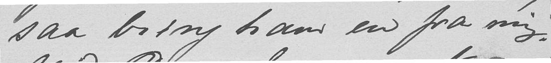saa bring ham en fra mig.
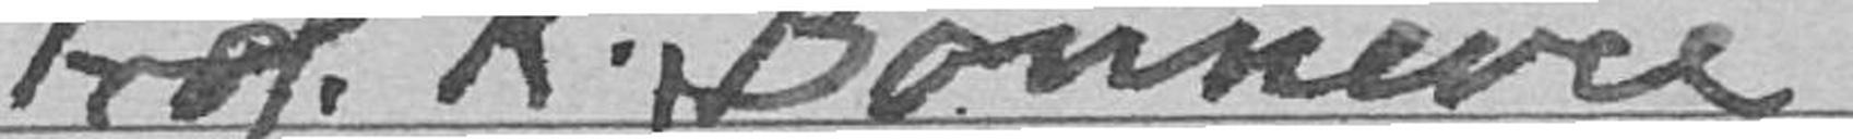Prof. K. Bonnevie
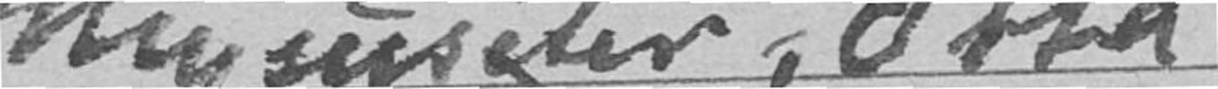Mysuseter, Otta
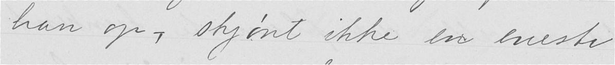han op -, skjønt ikke en eneste
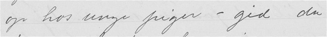op hos unge piger - gid du
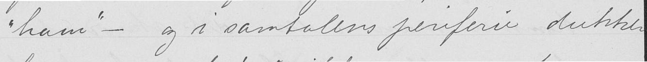"ham" - og i samtalens periferi dukker
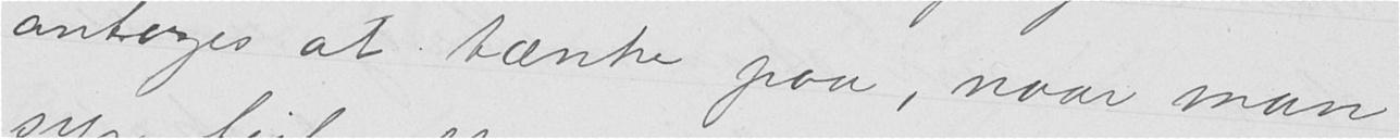antages at tænke paa, naar man
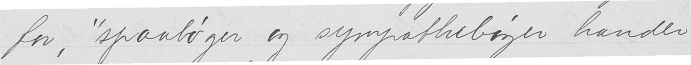for, "spaabøger og sympathibøger handler
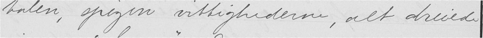talen, spøgen vittighederne, alt dreiede
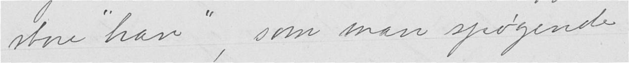store "han", som man spøgende
